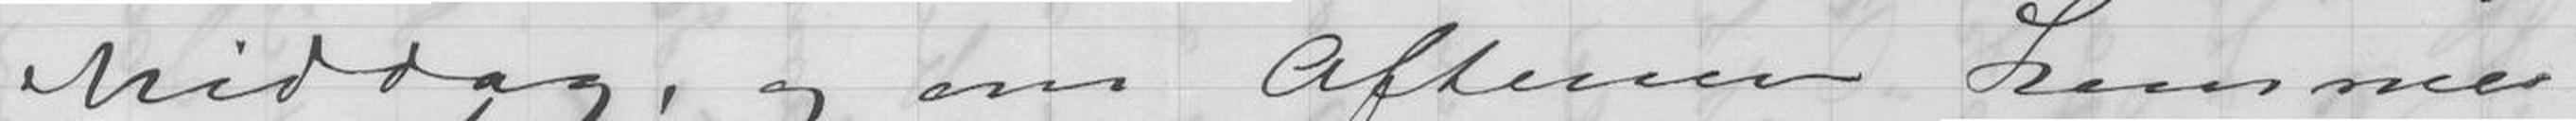Middag, og om Aftenen kommer
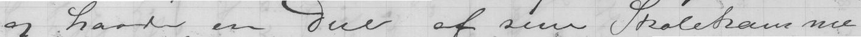og havde en Deel af sine Skolekamme
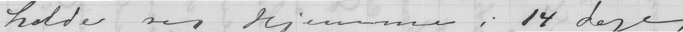holde sig Hjemme i 14 dage;
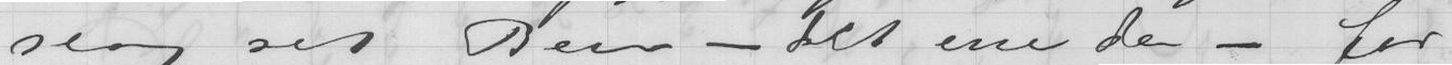slog sit Ben - det ene da - for
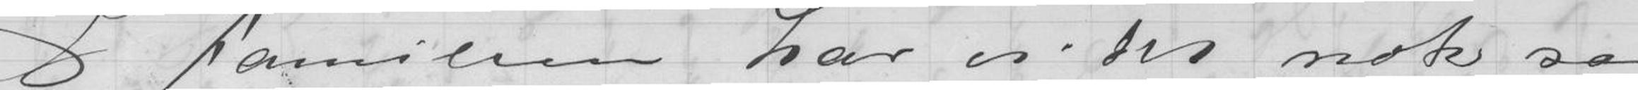I Familien har vi det nok sa
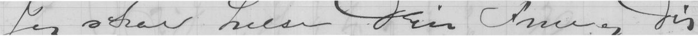Jeg skal hilse Din Frue og Dig
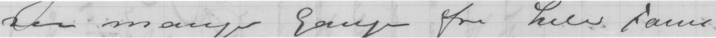saa mange Gange fra hele Fami
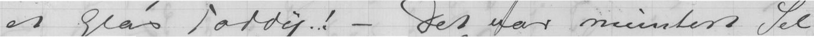et Glas Toddy! - Det var muntert Sel
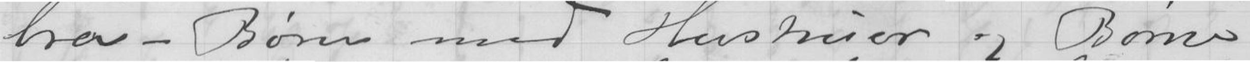bra - Børn med Hustruer og Børne
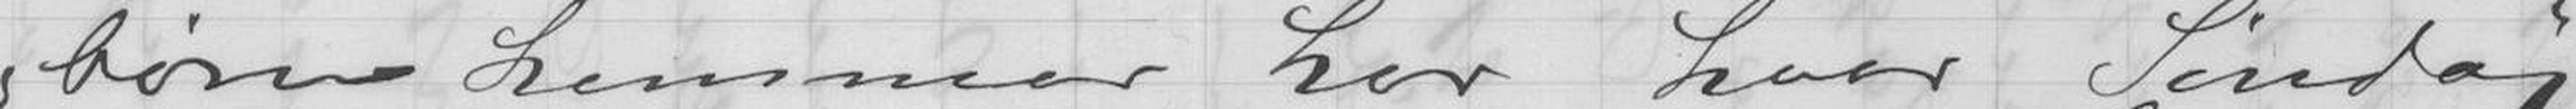børn kommer her hver Søndag
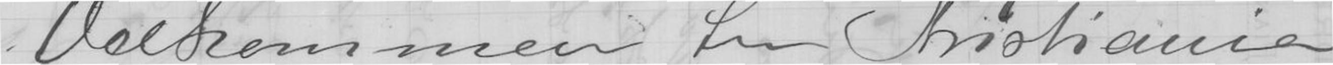Velkommen til Kristiania
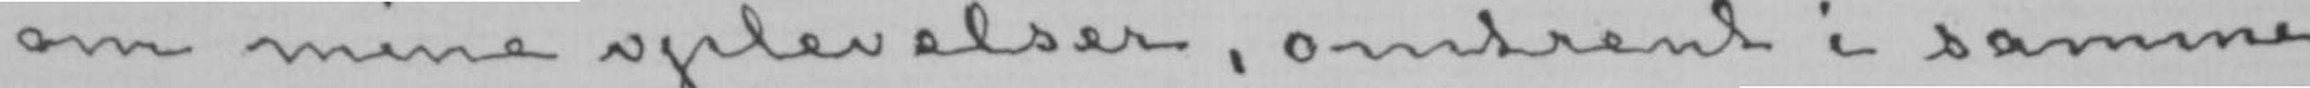om mine oplevelser, omtrent i samme
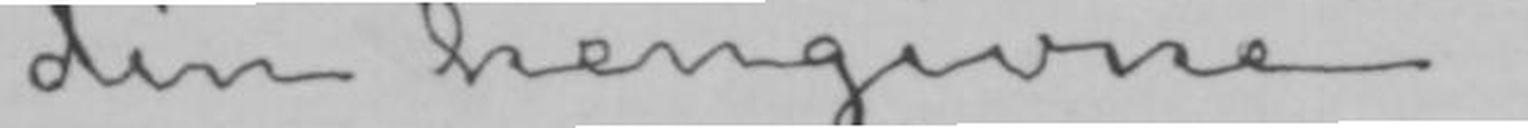din hengivne
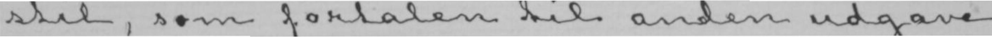stil, som fortalen til anden udgave
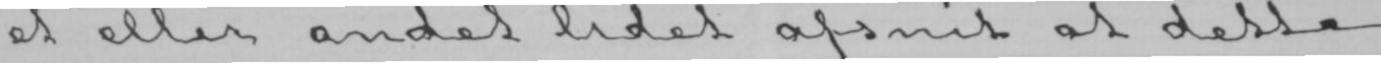et eller andet lidet afsnit af dette
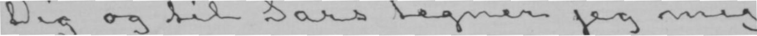Dig og til Sars tegner jeg mig
